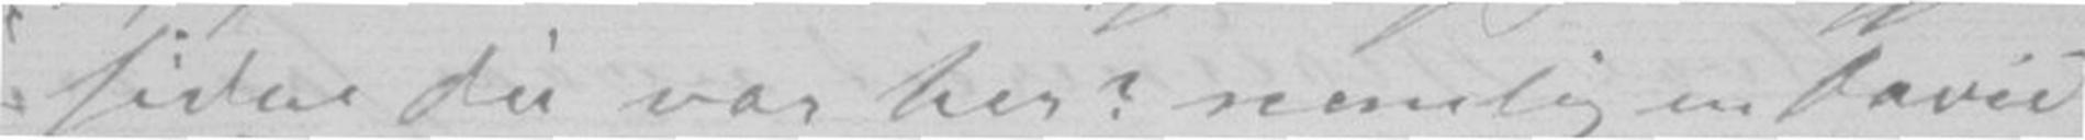siden du var her? nemligen David
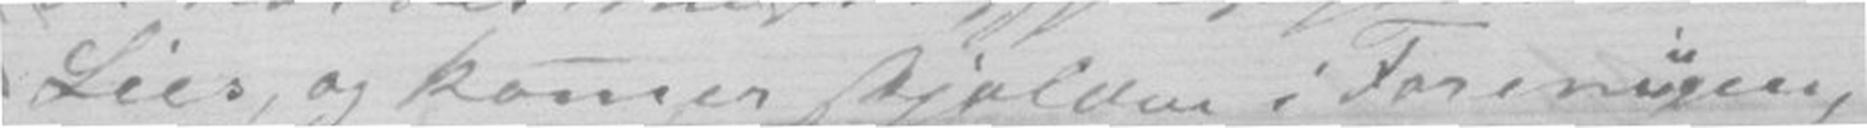Lies, og komer skjælden i Foreningen,
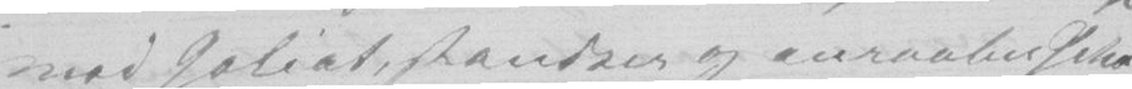mod Goliat, standser og anraaber Jeho-
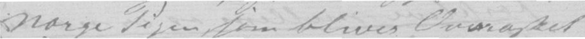Norge Pigen som bliver Overrasket
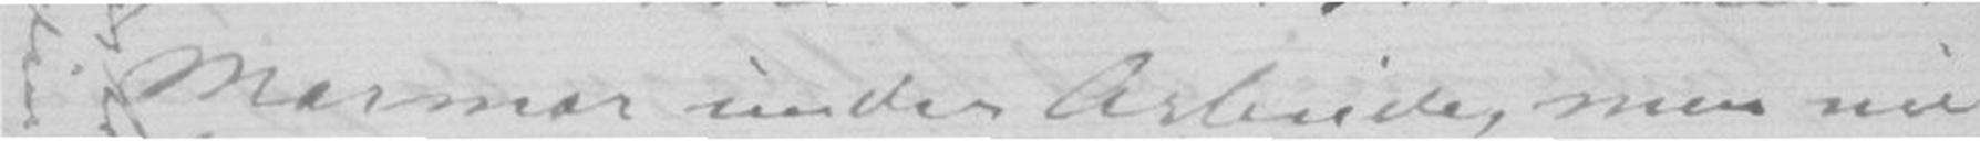Marmor under Arbeide, men nu
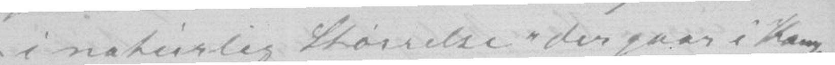i naturlig Størrelse der gaar i Kamp
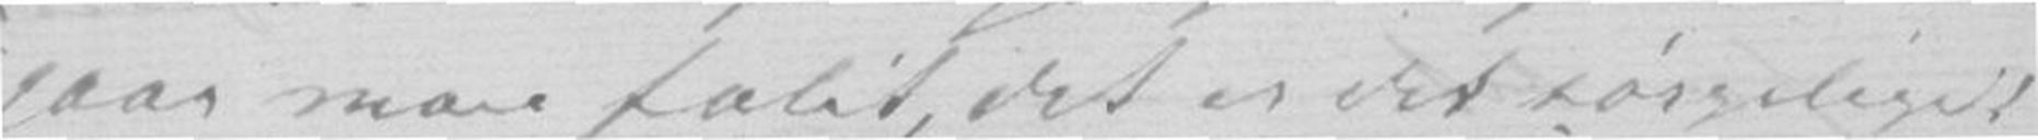gaar man falit, det er det sørgeligste.
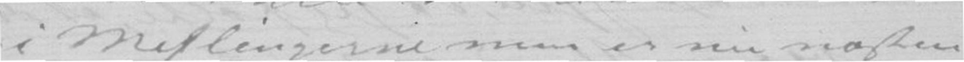i Meslingerne men er nu næsten
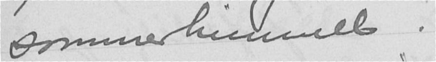sommerhimmel.
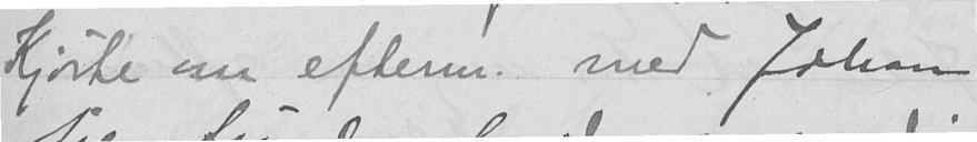Kjørte om efterm. med Johan
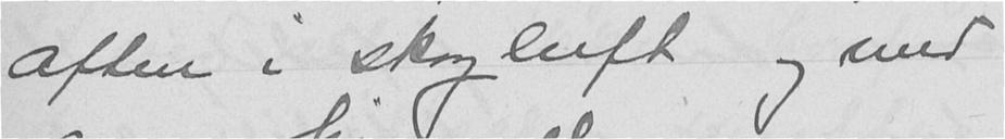aften i skogluft og med
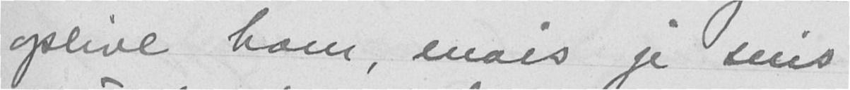oplive ham, mais je suis
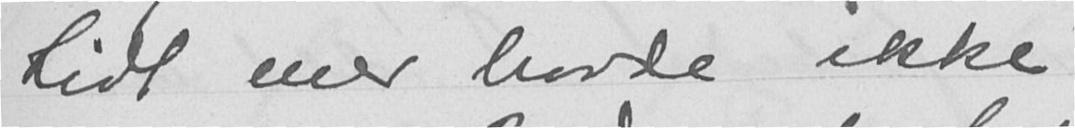Lidt mer havde ikke
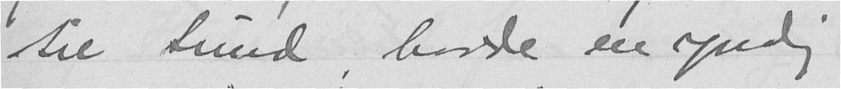til Sund, havde en yndig
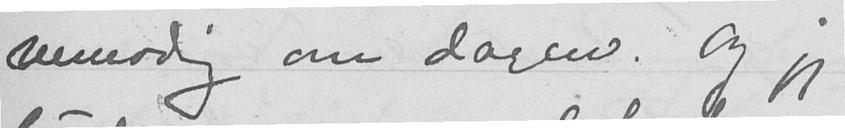vemodig om dagen. Og jeg
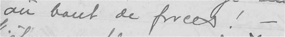au bout de forces! -
